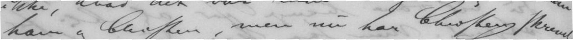ham og Christen, men nu har Christen skrevet
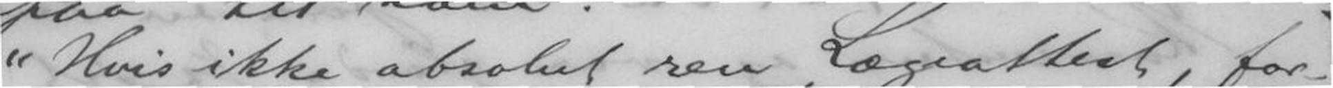"Hvis ikke absolut ren Lægeattest, for-
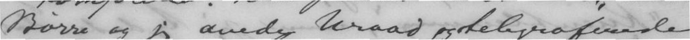Børre og jeg anede Uraad og telegraferede
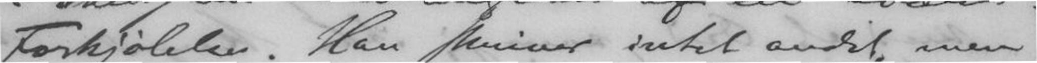Forkjølelse. Han skriver intet andet, men
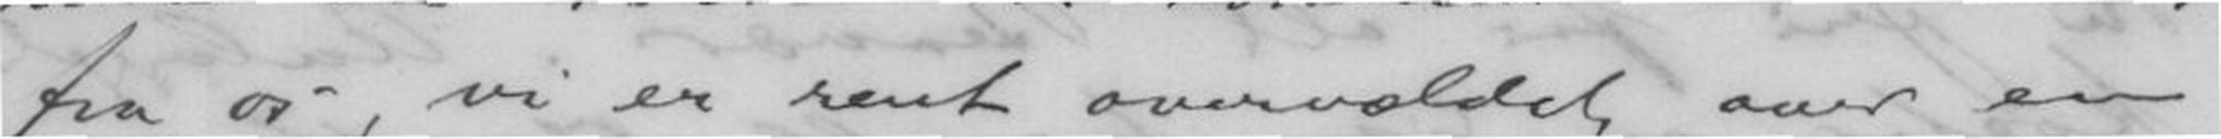fra os, vi er rent overvældet over en
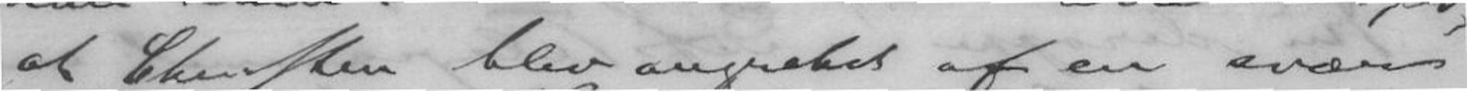at Christen blev angrebet af en svær
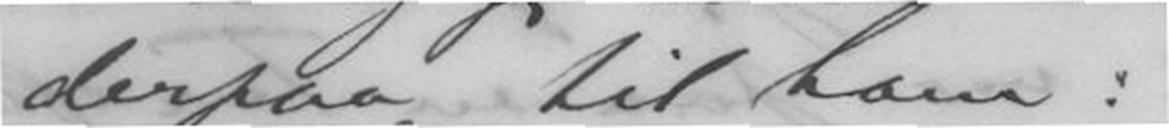derpaa til ham:
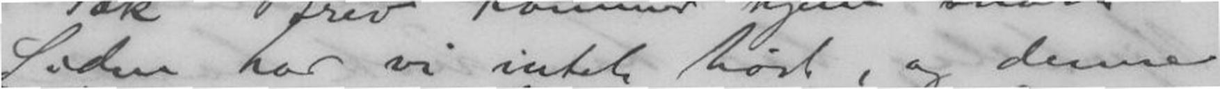Siden har vi intet hørt, og denne
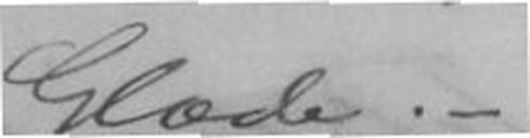Glæde. -
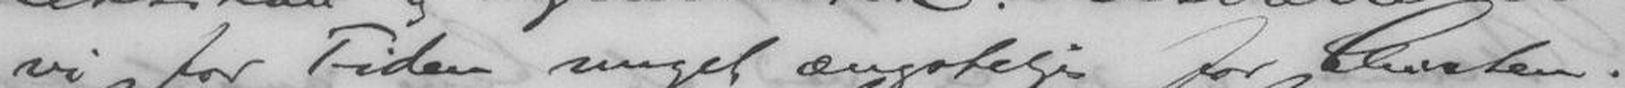vi for Tiden meget ængstelige for Christen.
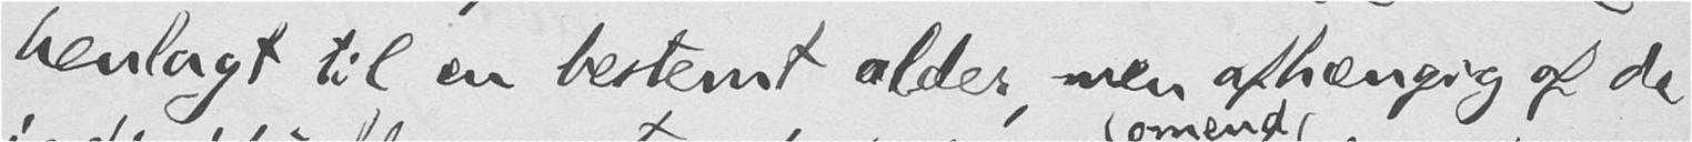henlagt til en bestemt alder, men afhængig af de
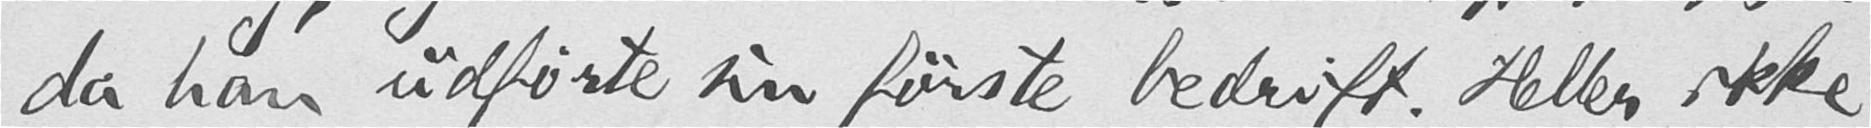da han udførte sin første bedrift. Heller ikke
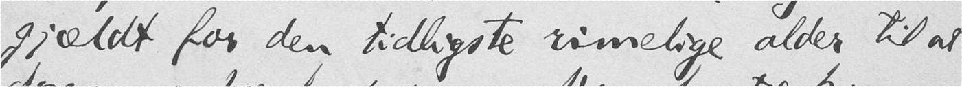gjældt for den tidligste rimelige alder til at
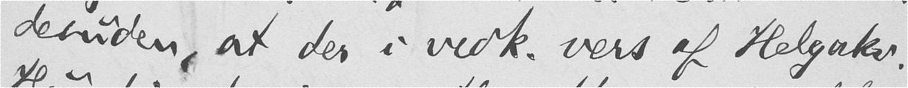desuden, at der i vedk. vers af Helgakv.
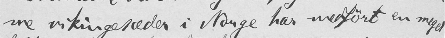me vikingesæder i Norge har medført en meget
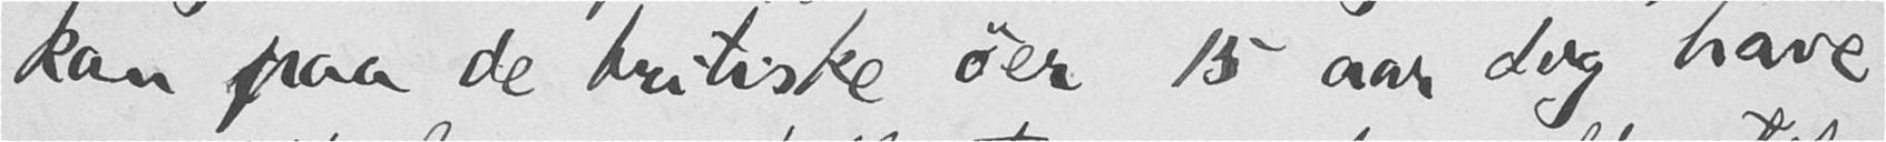kan paa de britiske øer 15 aar dog have
